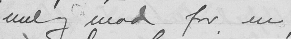mel og mad for en
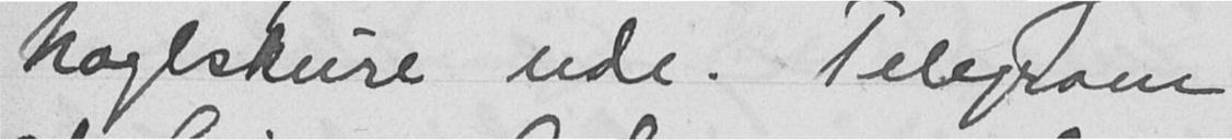haglskure ude. Telegram
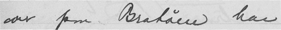over paa Bratøen har
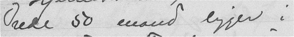rede 50 mand ligger i
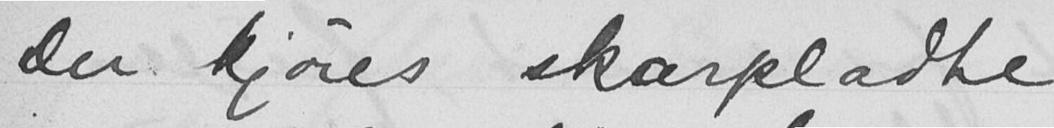Der kjøres skarpladte
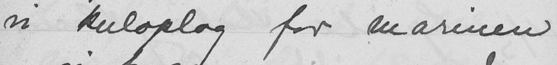vi kuloplag for marinen
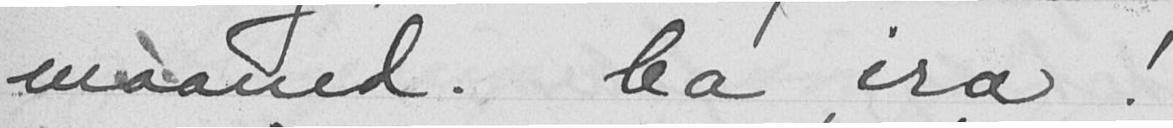maaned. Ca ira!
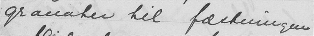granater til fæstningen
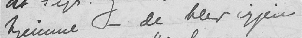hjemme - de blev igjen
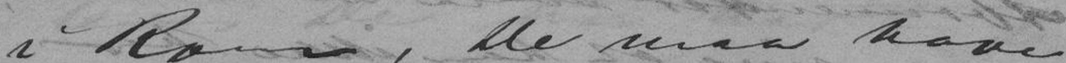i Rom, De maa have
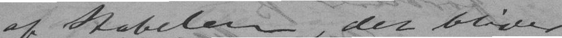af Stabelen, det bliver
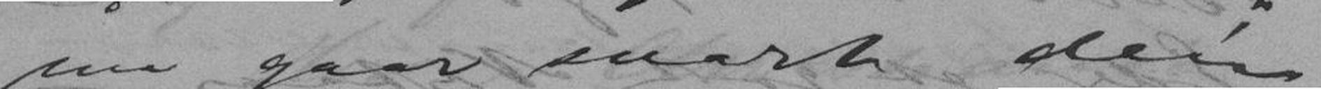nu gaar snart den
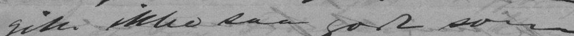gik ikke saa godt som
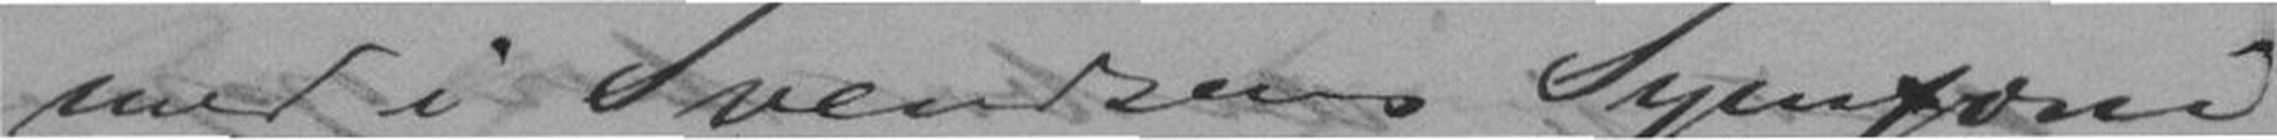med i Svendsens Synfoni,
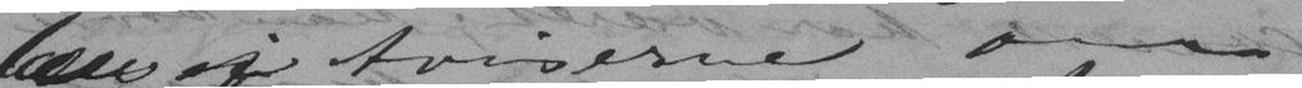læse i Aviserne om
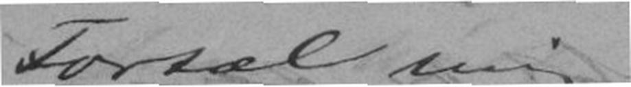Fortæl mig
